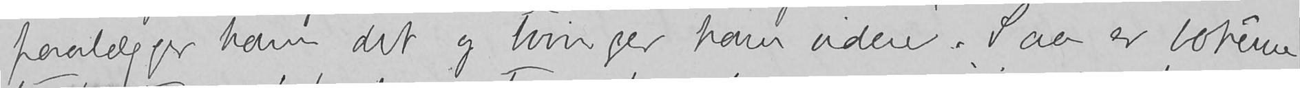paalægger ham det og tvinger ham videre. Saa er bohéme
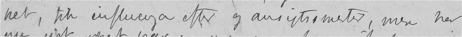ket, fik influenza efter og ansigtssmerter, men har
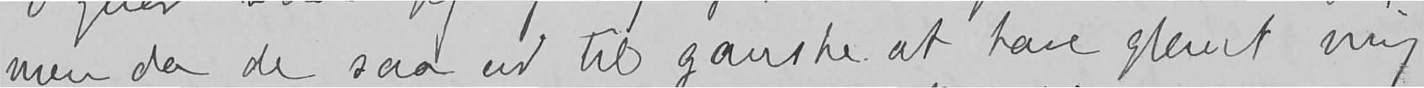men da de saa ud til ganske at have glemt mig,
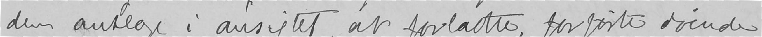den anklage i ansigtet, at forladte, forførte døende
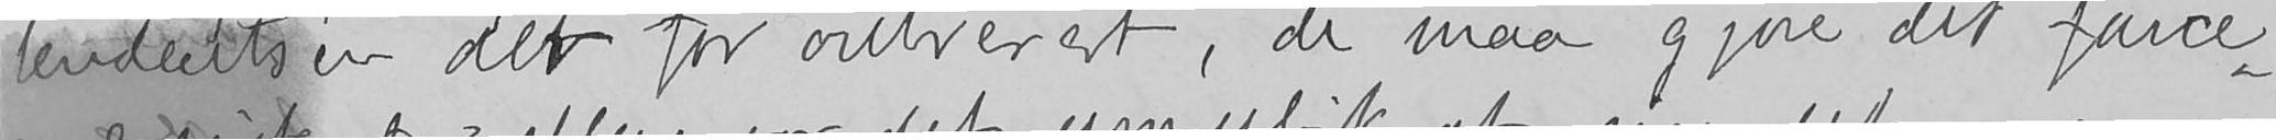tendentsen det for ontreret, de maa gjøre det farce-
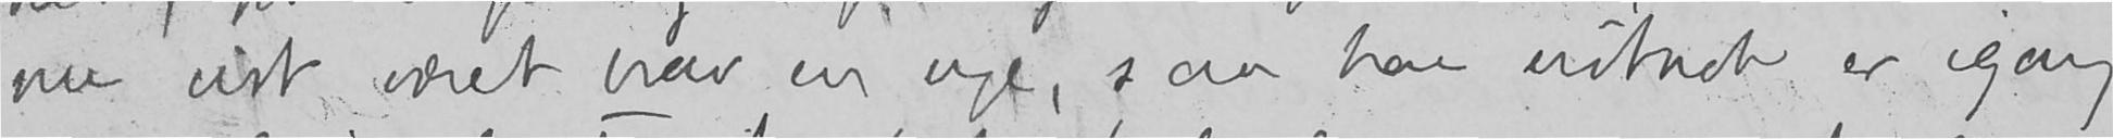nu vist været brav en uge, saa han vistnok er igang
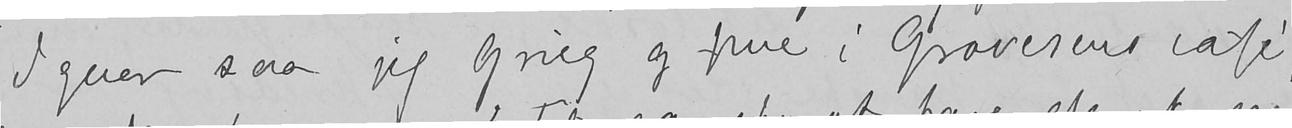Igaar saa jeg Grieg og frue i Gravesens café,
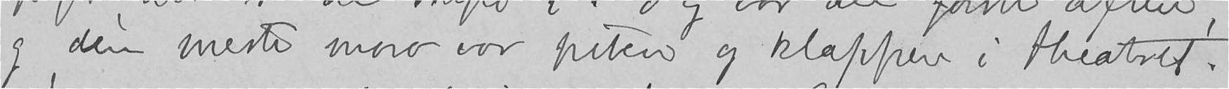og den meste moro var piben og klappen i theatret.
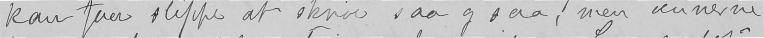kan faa slippe at skrive saa og saa, men vennerne
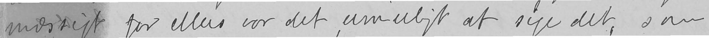mæssigt for ellers var det umuligt at sige det, som
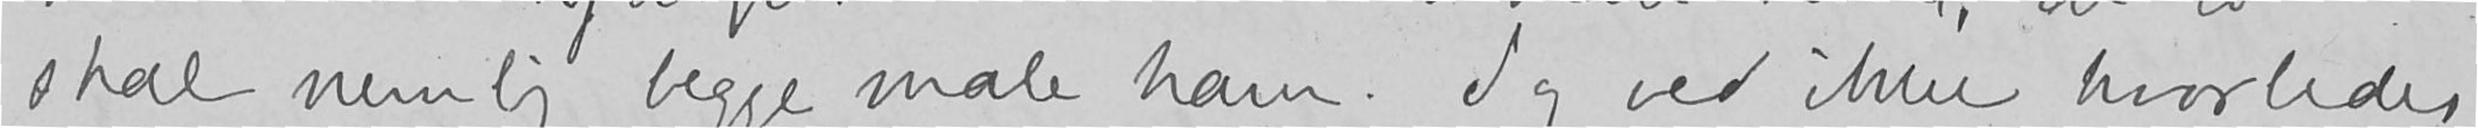skal nemlig begge male ham. Jeg ved ikke hvorledes
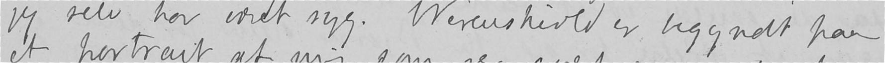jeg selv har været syg. Werenskiold er begyndt paa
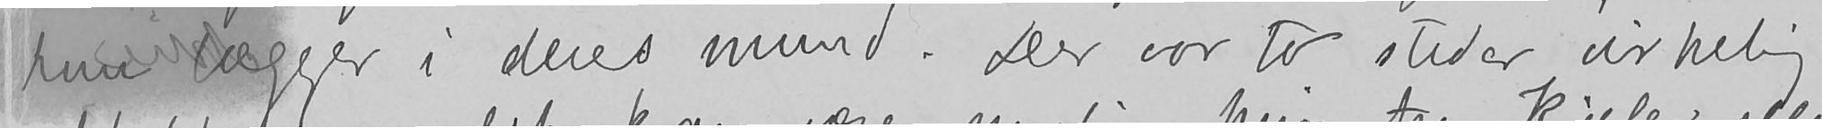hun lægger i deres mund. Der var to steder virkelig
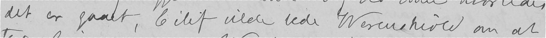det er gaaet, Eilif vilde bede Werenskiold om at
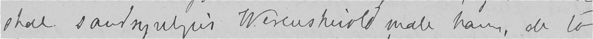skal sandsynligvis Werenskiold male ham, de to
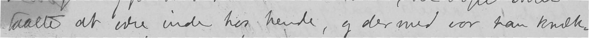taalte at være inde hos hende, og dermed var han knæk-
